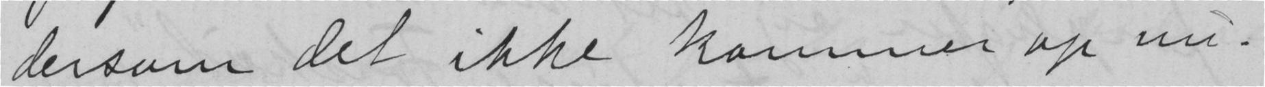dersom det ikke kommer op nu.
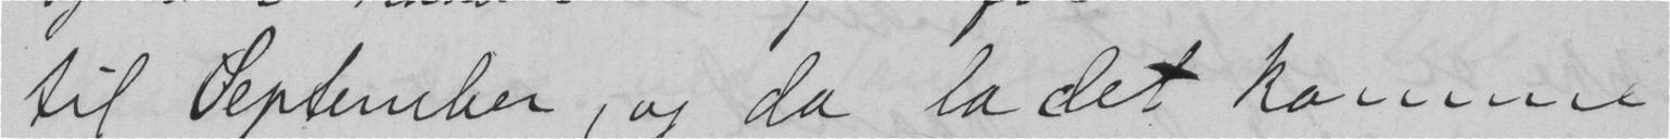til September, og da la det komme
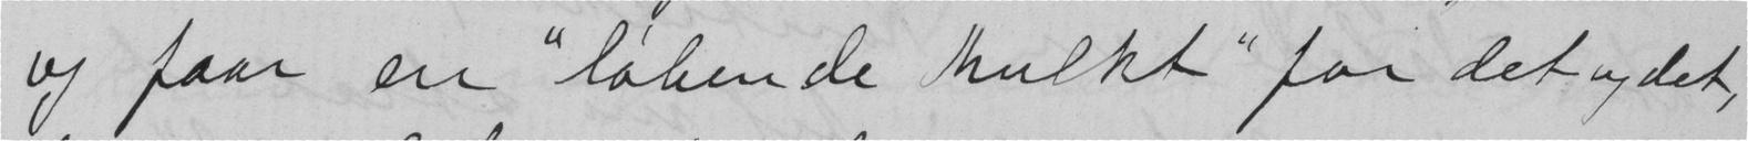og faar en "løbende Mulkt" for det og det,
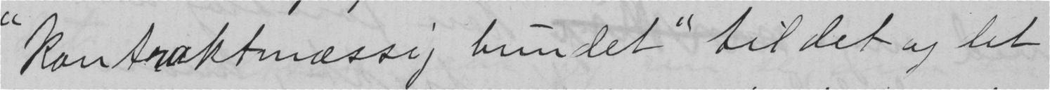"kontraktmæssig bundet" til det og det
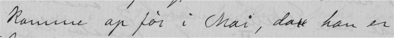komme op før i Mai, da han er
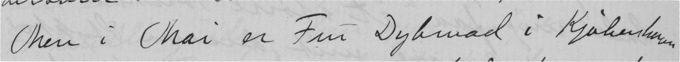Men i Mai er Fru Dybwad i Kjøbenhavn
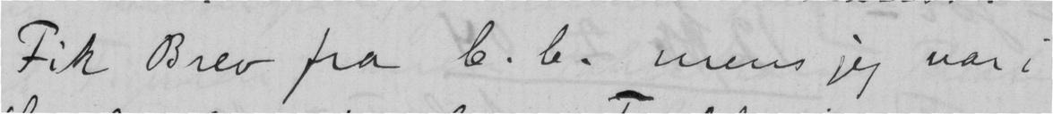Fik Brev fra b. b. mens jeg var i
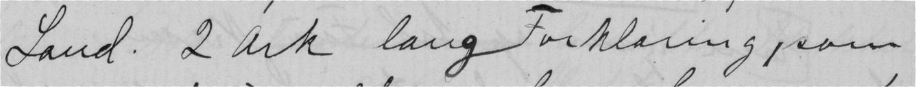Land. 2 Ark lang Forklaring, som
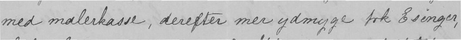med malerkasse, derefter mer ydmyge frk Esinger,
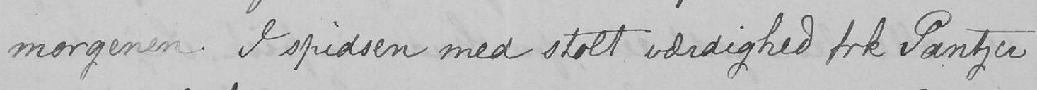morgenen. I spidsen med stolt værdighed frk Pantzer
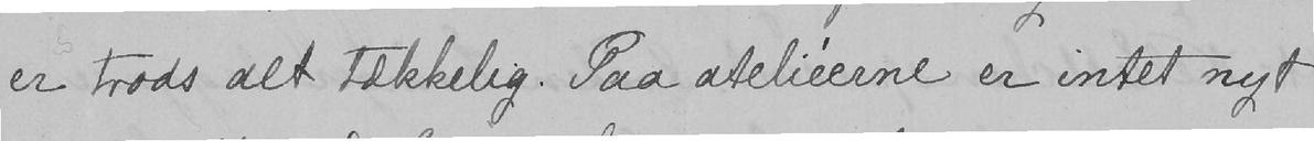er trods alt tækkelig. Paa ateliéerne er intet nyt
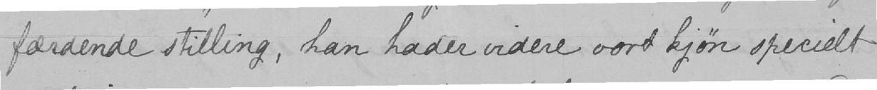færdende stilling, han hader videre vort kjøn specielt
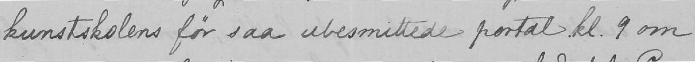kunstskolens før saa ubesmittede portal kl. 9 om
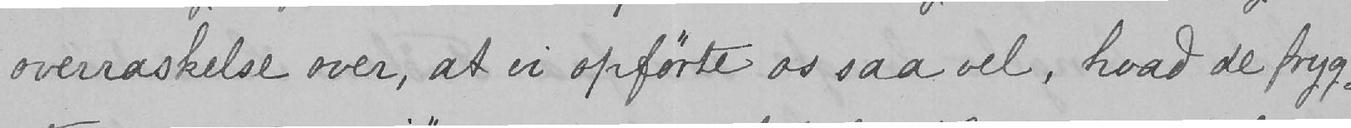overraskelse over, at vi opførte os saa vel, hvad de fryg-
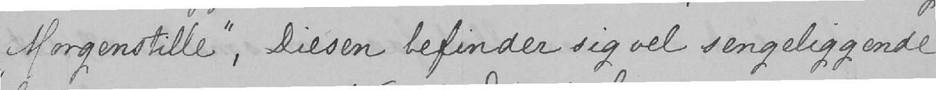«Morgenstille», Diesen befinder sig vel sengeliggende
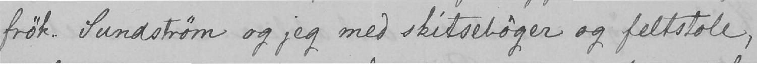frøk. Sundstrøm og jeg med skitsebøger og feltstole,
